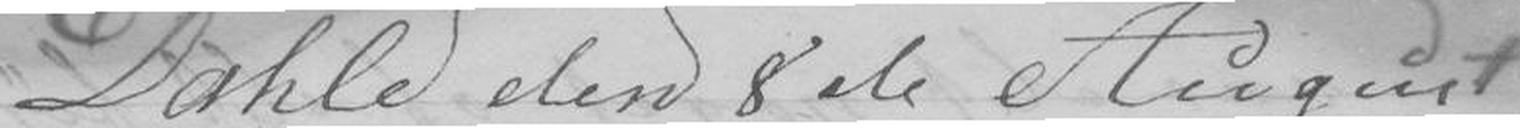Dahle den 8 de August
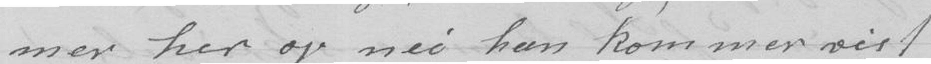mer her op nei han kommer vist
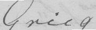Grieg
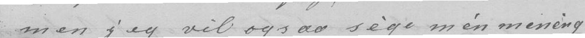men jeg vil ogsaa sige min mening
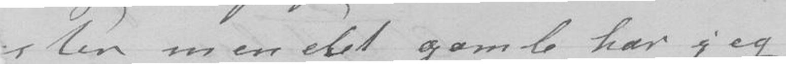ter men det gamle har jeg
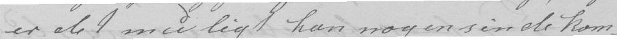er det muligt han nogensinde kom-
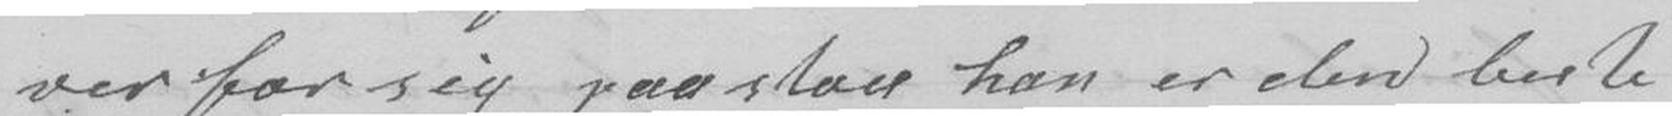ver for sig paastaa han er den beste
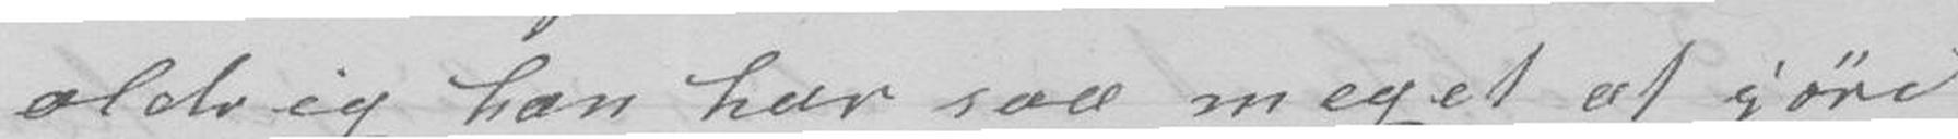aldrig han har saa meget at jøre
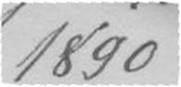1890
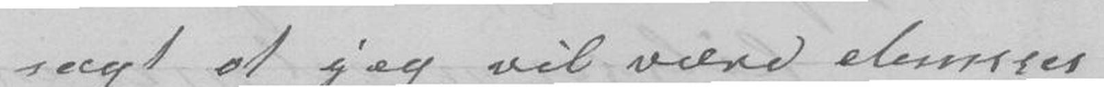sagt at jeg vil være demsses
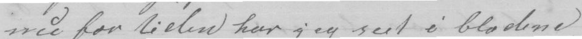nu for tiden har jeg seet i bladene
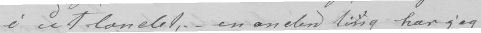i utlandet, - - en anden ting har jeg
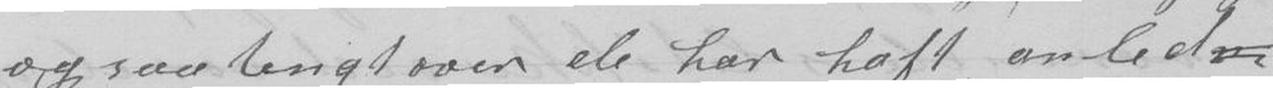ogsaa tengt over de har haft anled-
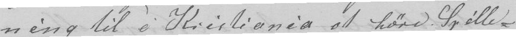ning til i Kristiania at høre Spille-
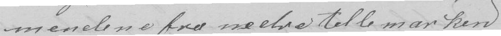mendene fra nedre tellemarken
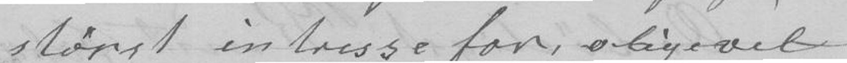størst intresse for, aligevel
